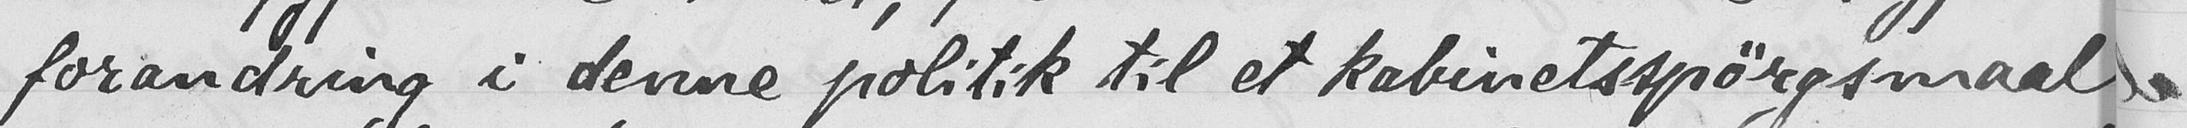forandring i denne politik til et kabinetsspørgsmaal og
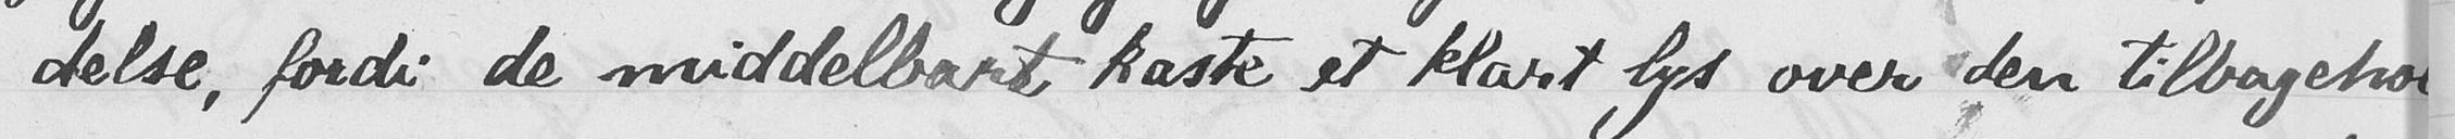delse, fordi de middelbart kaste et klart lys over den tilbageholden-
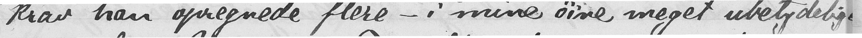krav han opregnede flere - i mine øine meget ubetydelige -
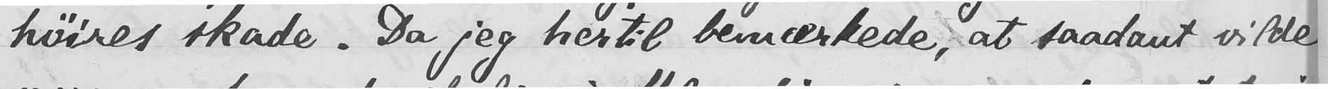høires skade. Da jeg hertil bemærkede, at saadant vilde
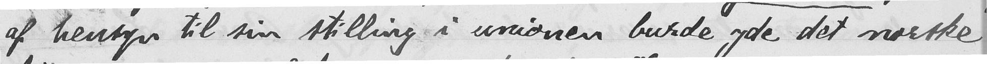af hensyn til sin stilling i unionen burde yde det norske
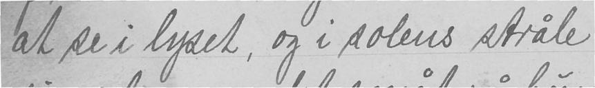at se i lyset, og i solens stråle
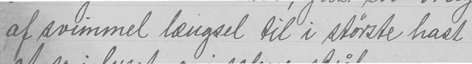af svimmel længsel til i største hast
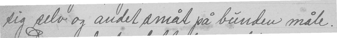sig selv og andet småt på bunden måle.
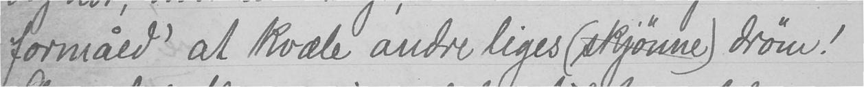formåed' at kvæle andre liges (skjønne) drøm!
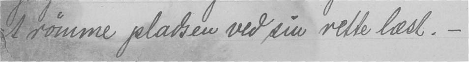t rømme pladsen ved sin rette læst. -
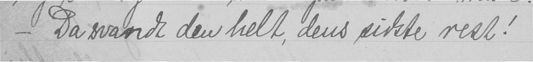- Da svandt den helt, dens sidste rest!
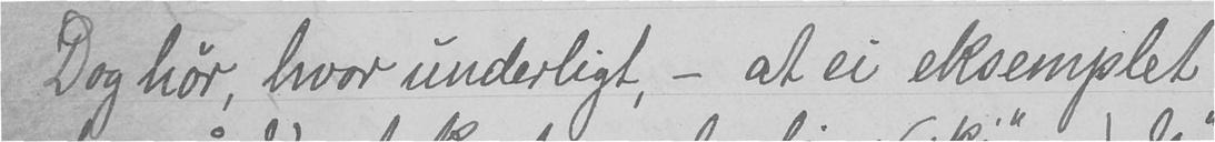Dog hør, hvor underligt, - at ei eksemplet
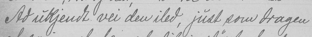Ad ukjendt vei den iled, just som dragen
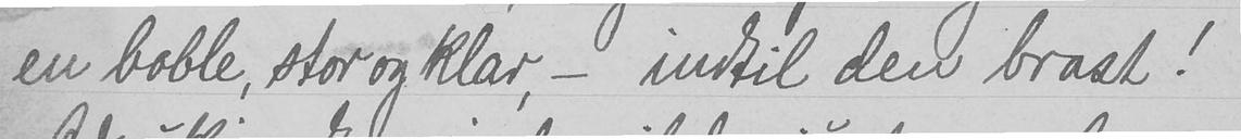en boble, stor og klar, - indtil den brast!
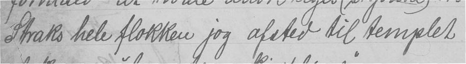Straks hele flokken jog afsted til templet
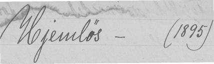Hjemløs - (1895)
